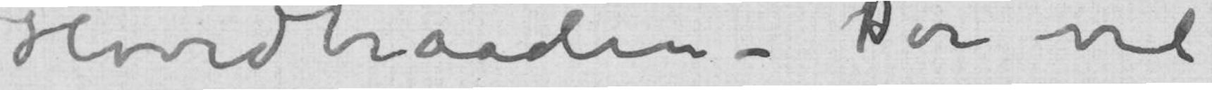Hovedtraaden – Der vil
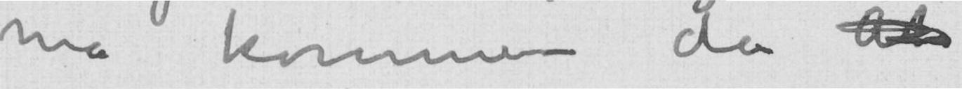må komme da at
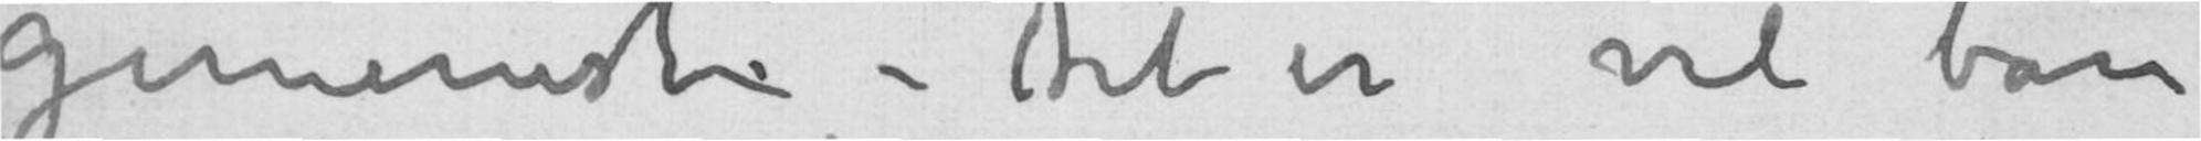gemeneste – Det er vel bare
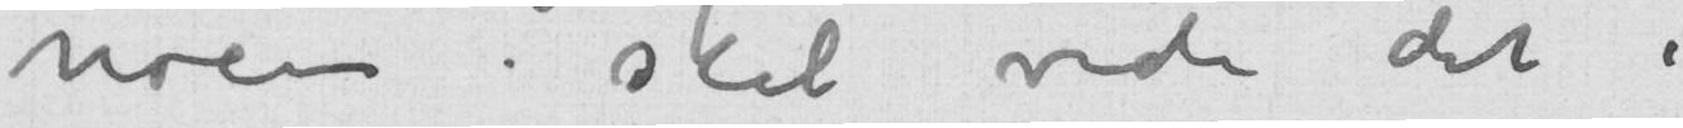noen skal vide det i
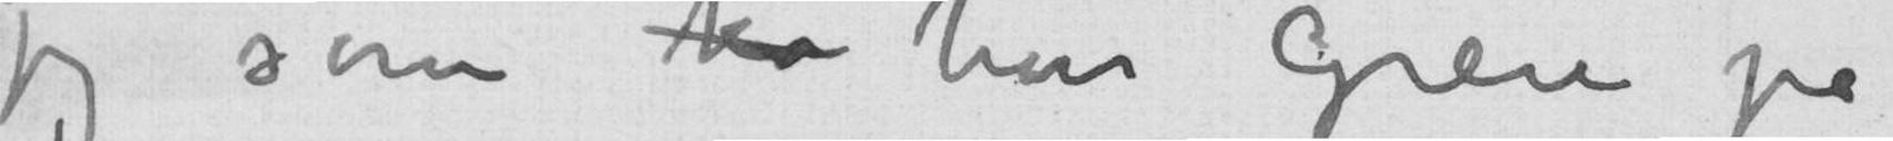jeg som ka har Greie på
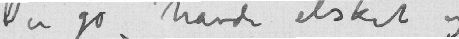Du jo havde elsket og
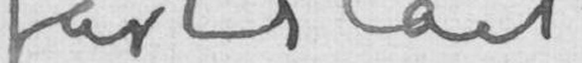fastslået
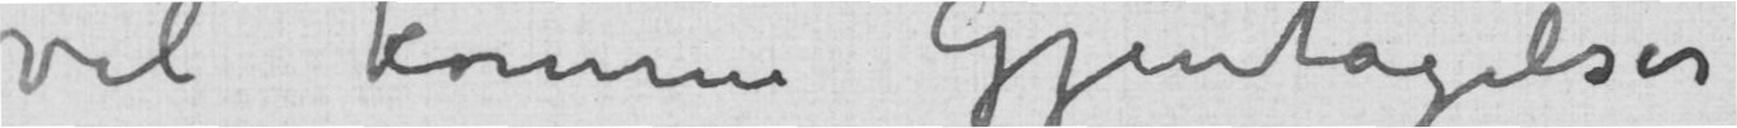vel komme Gjentagelser
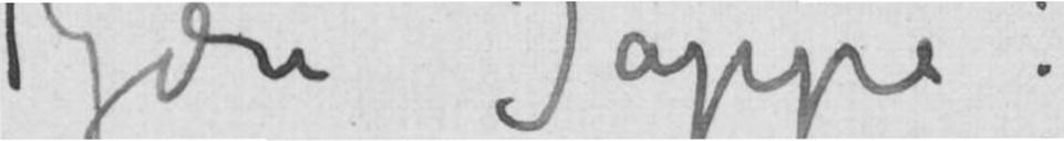Kjære Jappe!
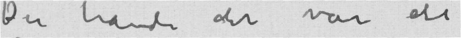Du havde det var det
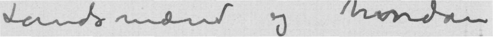Landsmænd og hvordan
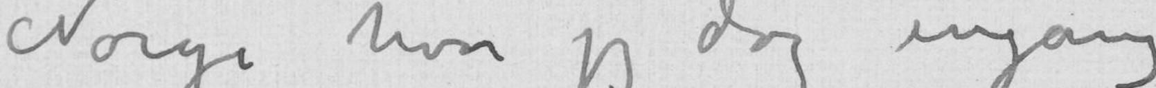Norge hvor jeg dog engang
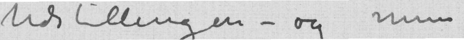Udstillingen – og mine
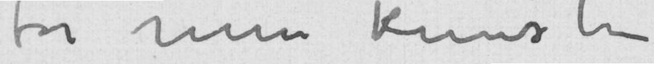for min Kunst
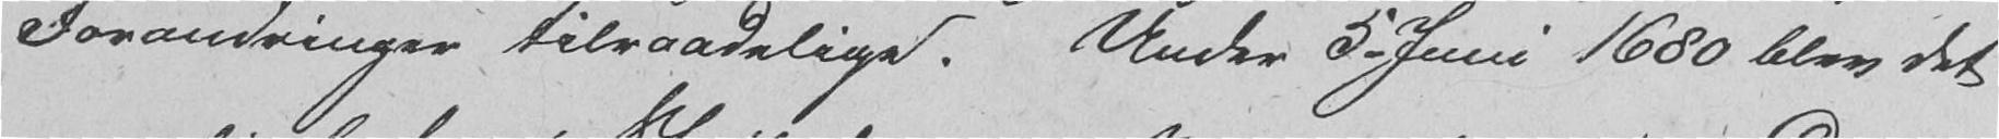Forandringer tilraadelige. Under 5. Juni 1680 blev det
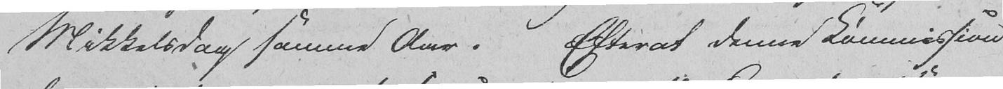Mikkelsdag samme Aar. Efterat denne Kommission
